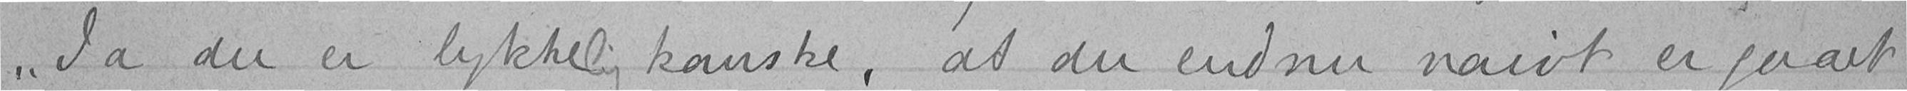"Ja du er lykkelig kanske, at du endnu naivt er gaaet
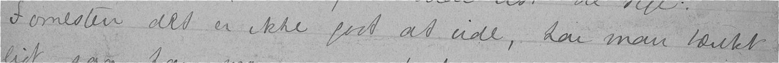Forresten det er ikke godt at vide, har man tænkt
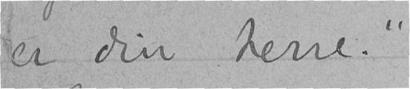er din herre."
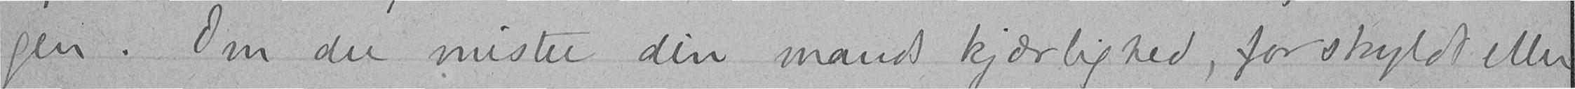gen. Om du mister din mands kjærlighed, forskyldt eller
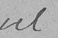vel
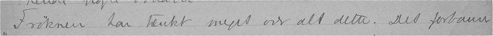"Frøknen har tænkt meget over alt dette. Det forbauser
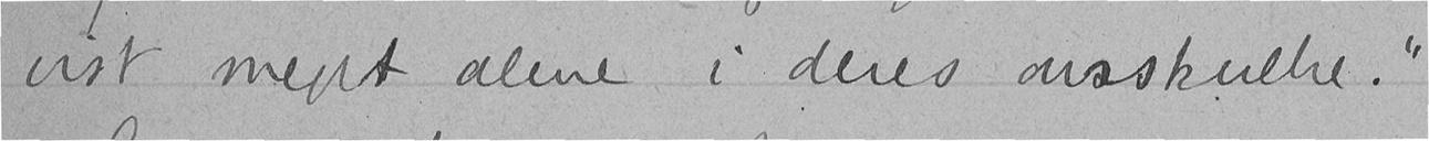vist meget alene i deres anskuelse."
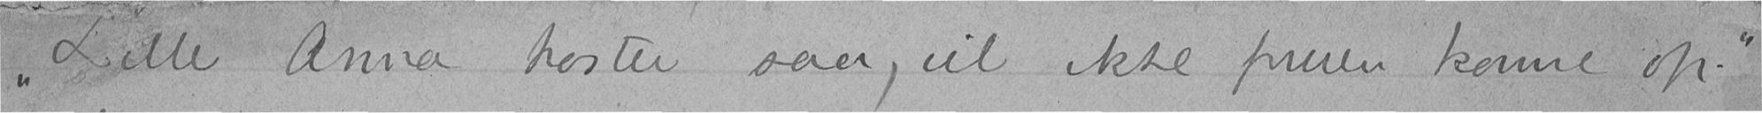"Lille Anna hoster saa, vil ikke fruen komme op."
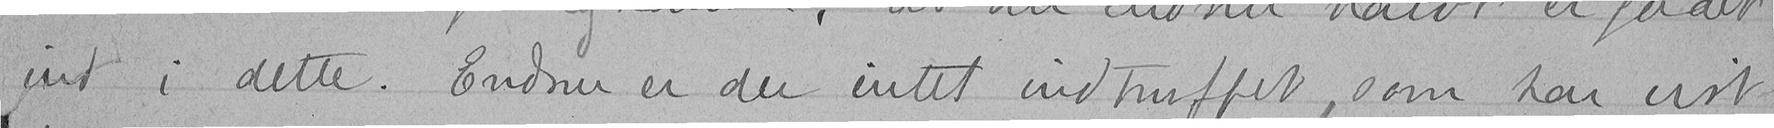ind i dette. Endnu er der intet indtruffet, som har vist
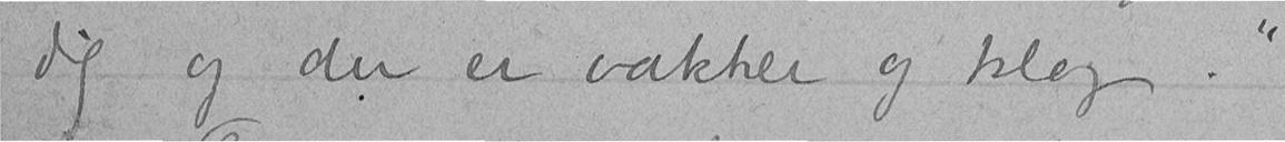dig og du er vakker og klog."
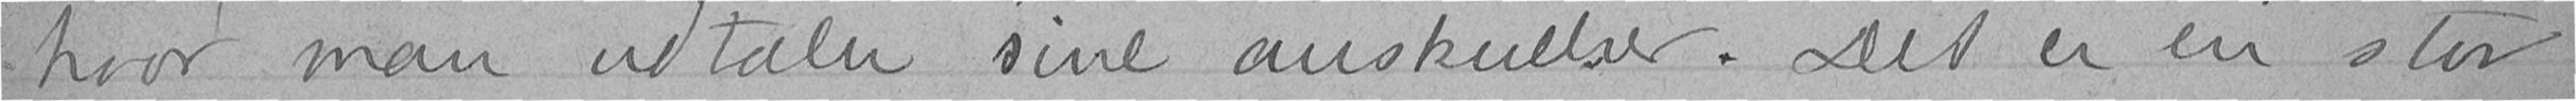hvor man udtaler sine anskuelser. Det er en stor
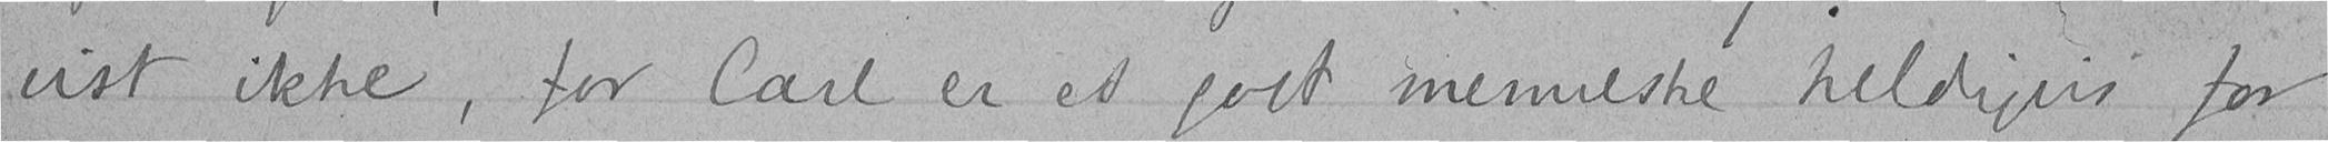vist ikke, for Carl er et godt menneske heldigvis for
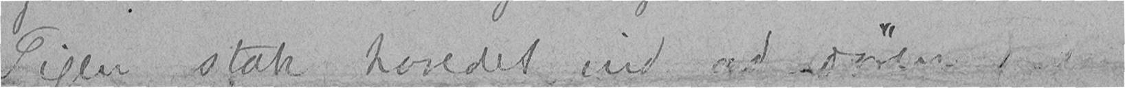Pigen stak hovedet ind ad døren
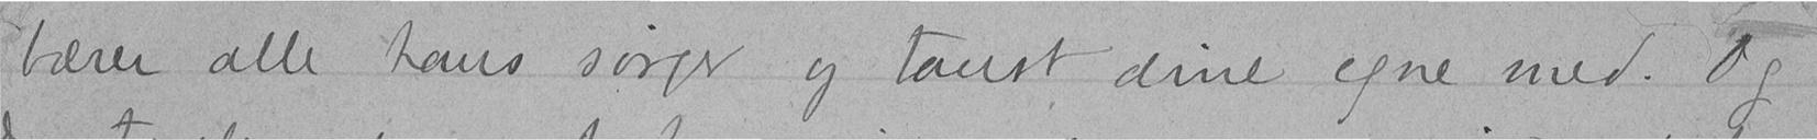bærer alle hans sorger og taust dine egne med. Og
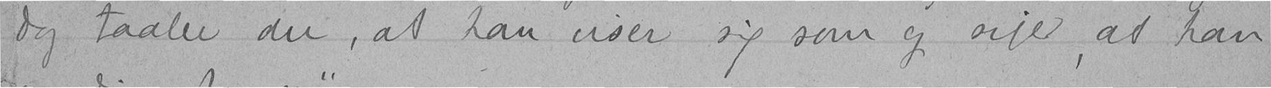dog taaler du, at han viser sig som og siger, at han
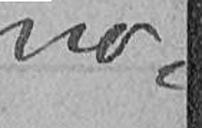no-
